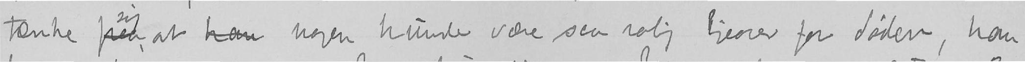tænke paa, at han nogen kunde være saa rolig ligeover for døden, han
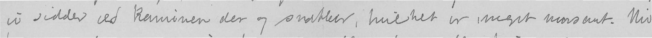vi sidder ved kaminen der og snakker, hvilket er meget morsomt. Nu
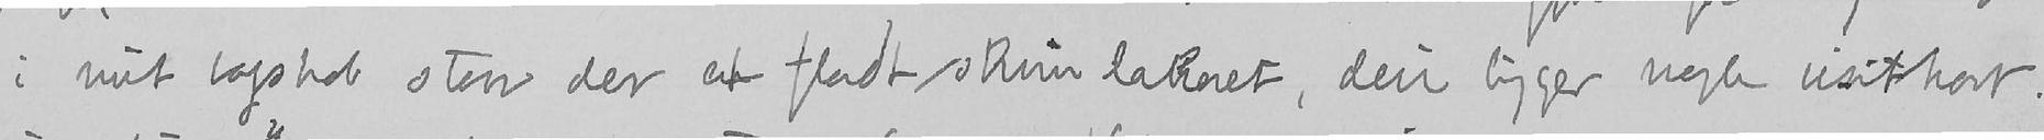i mit bogskab staar der ent fladt skrin lakeret, deri ligger nogle visitkort.
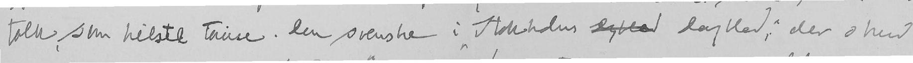folk, som hilste tause. Den svenske i «Stokholm Dgblad Dagblad», der skrev
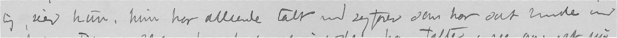lig, sier hun, hun har allerede talt med sagfører som har sat hende ind
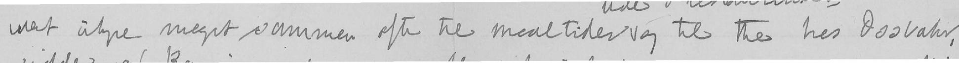været uhyre meget sammen, ofte til maaltider og til the hos Ossbahr,
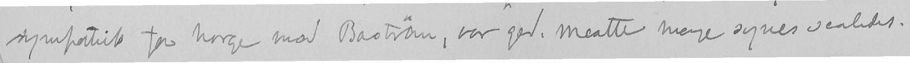sympathisk for Norge mod Boström, var god, maatte mange synes saaledes.
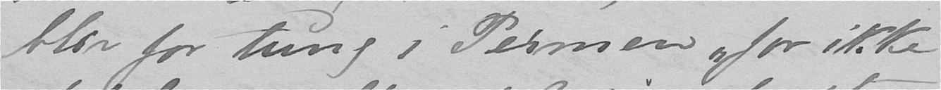blir for tung i Permen "for ikke
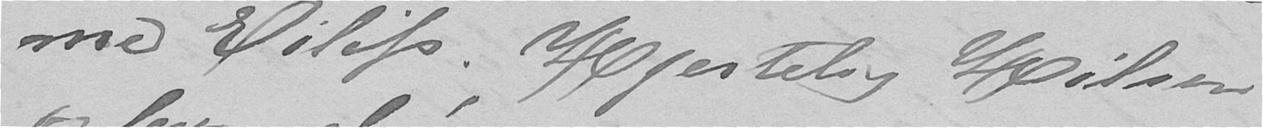med Eilif. Hjertelig Hilsen
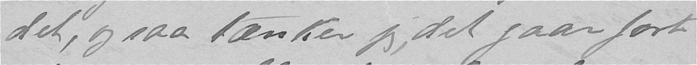det, og saa tænker jeg, det gaar fort.
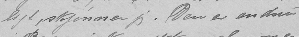ligt, skjønner jeg. Den er endnu
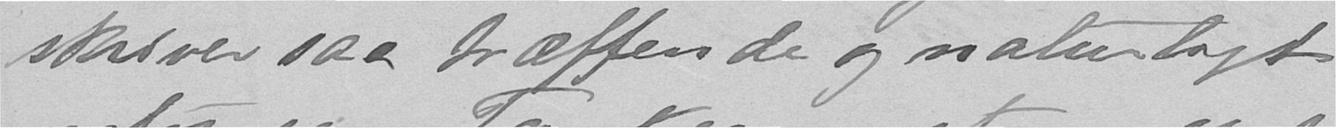skriver saa træffende og naturligt
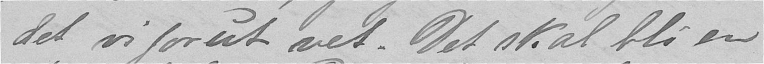det vi forut vet. Det skal bli en
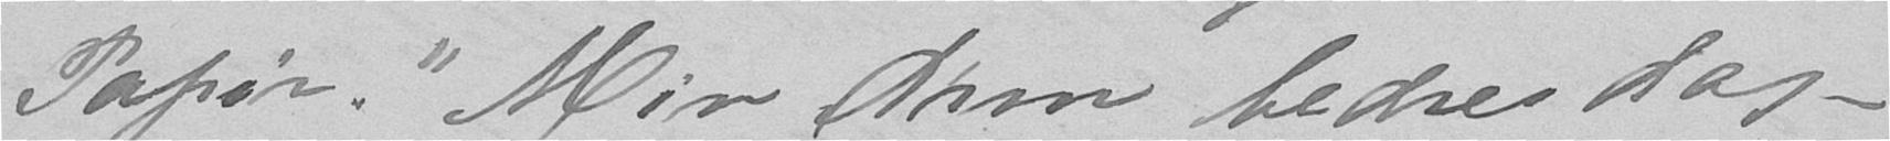Papir." Min Arm bedres dag-
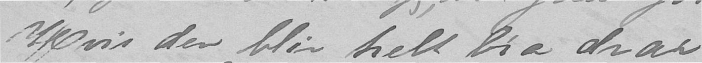Hvis den blir helt bra drar
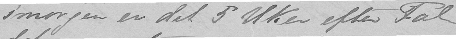i morgen er det 5 Uker efter Fal-
